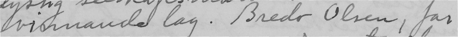vinnande lag. Bredo Olsen, far
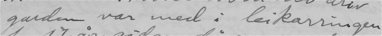garden var med i leikarringen
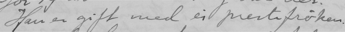Han er gift med ei prestefröken.
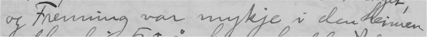og Fremming var mykje i den heimen
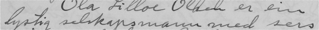lystig selskapsmann med særs
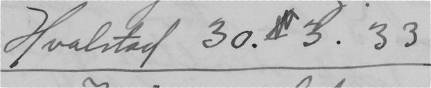Hvalstad 30.2.33
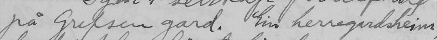på Grefsen gard. Ein herregardsheim
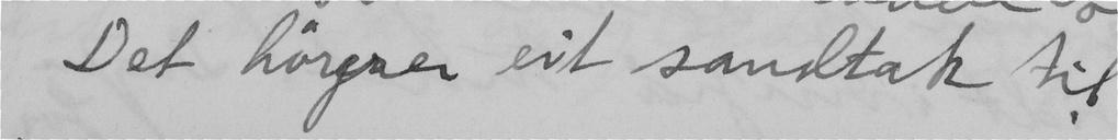Det hörer eit sandtak til.
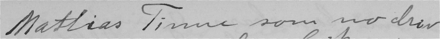Mathias Tinne som no driv
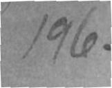196.
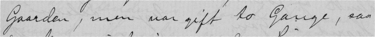Gaarden, men var gift to Gange, saa
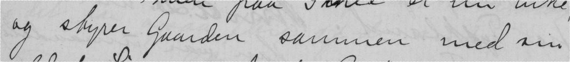og styrer Gaarden sammen med sin
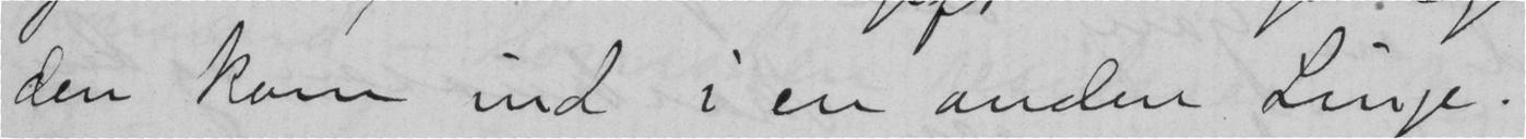den kom ind i en anden Linje.
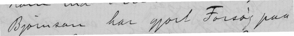Bjørnson har gjort Forsøg paa
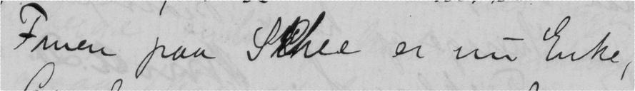Fruen paa Schee er nu Enke,
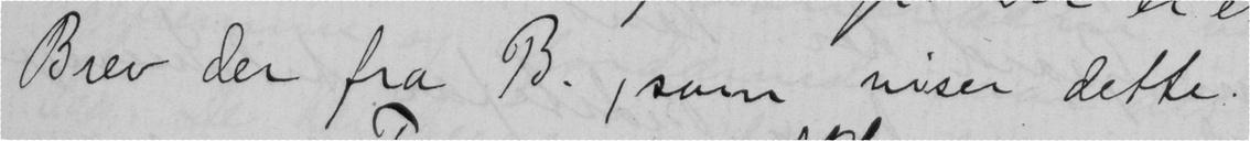Brev der fra B., som viser dette.
